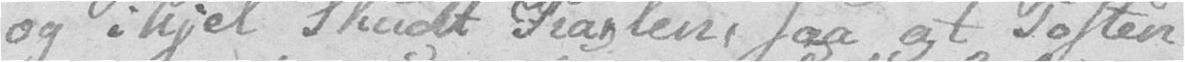og ihjel Skudt Karlen, saa at Posten
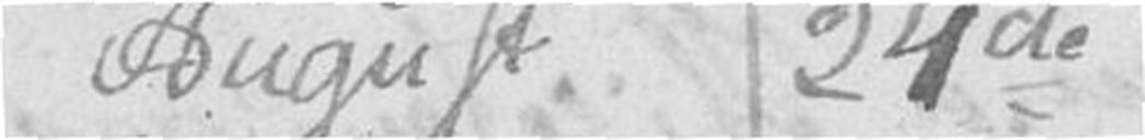August 24de
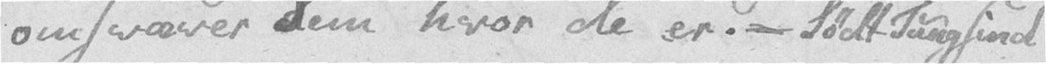omsværer dem hvor de er. – Sødt Tungsind
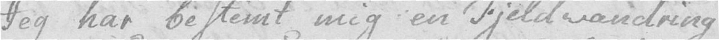Jeg har bestemt mig en Fjeldvandring
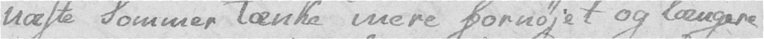næste Sommer tænke mere fornøjet og længere
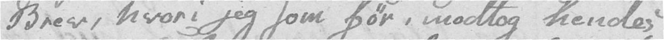Brev, hvori jeg som før, modtog hendes
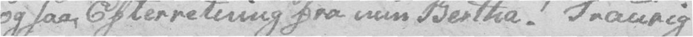ogsaa Efterretning fra min Bertha! Traurig
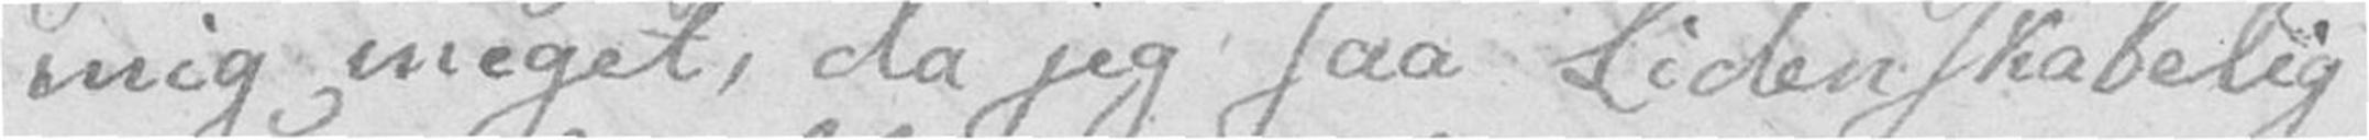mig meget, da jeg saa Lidenskabelig
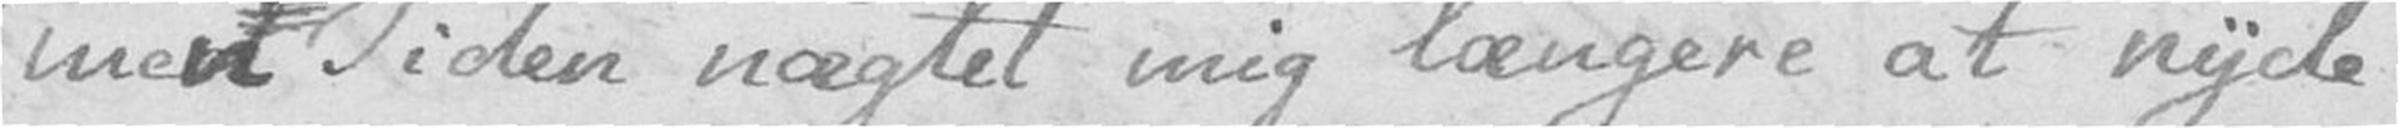men Tiden nægtet mig længere at nyde
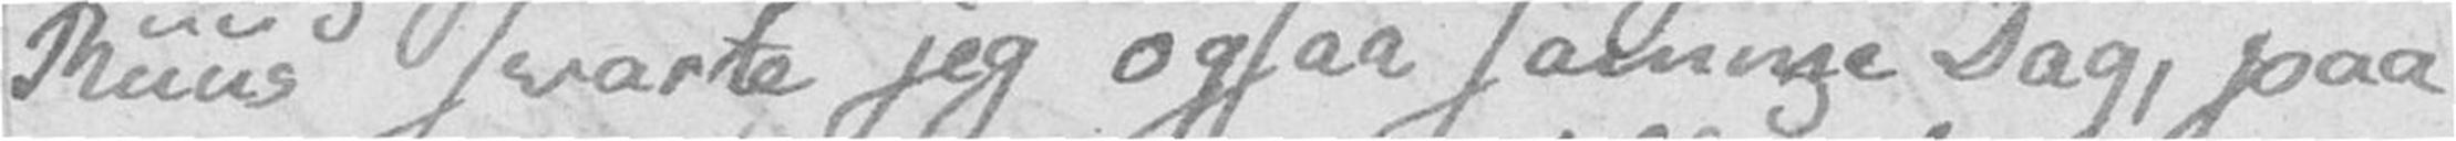Ruus svarte jeg ogsaa samme Dag, paa
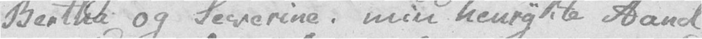Bertha og Severine! min henrykte Aand
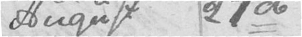August 27de
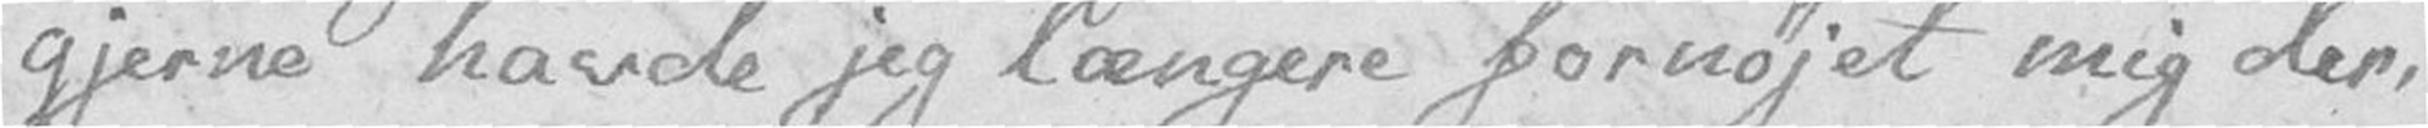gjerne havde jeg længere fornøjet mig der,
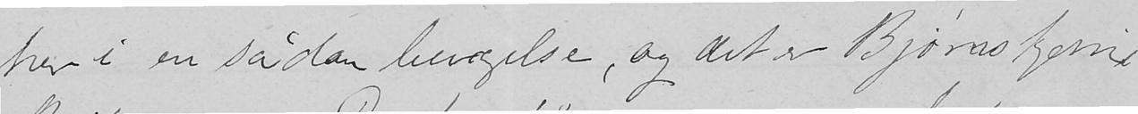her i en sådan bevægelse, og det er Bjørnstjerne
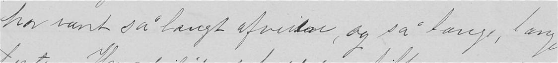har været så langt afveien, og så længe, længe
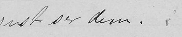just ser dem.
